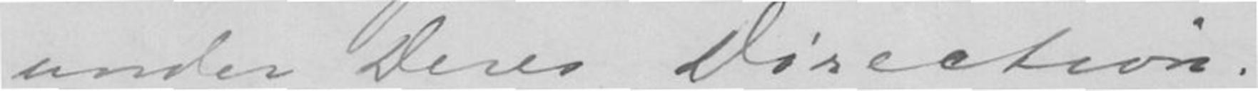under Deres Direction.
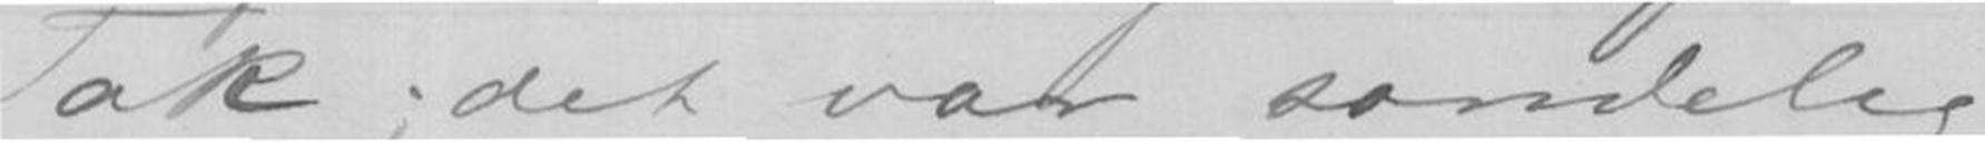Tak; det var sandelig
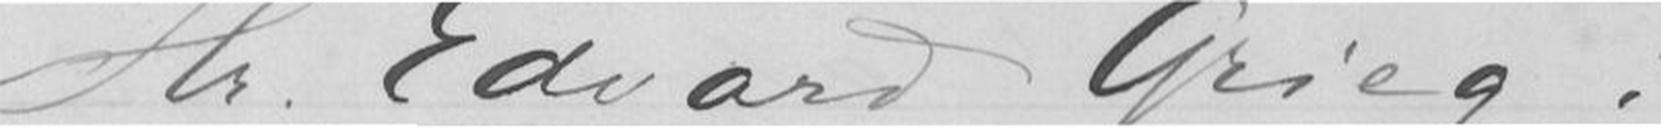Hr. Edvard Grieg!
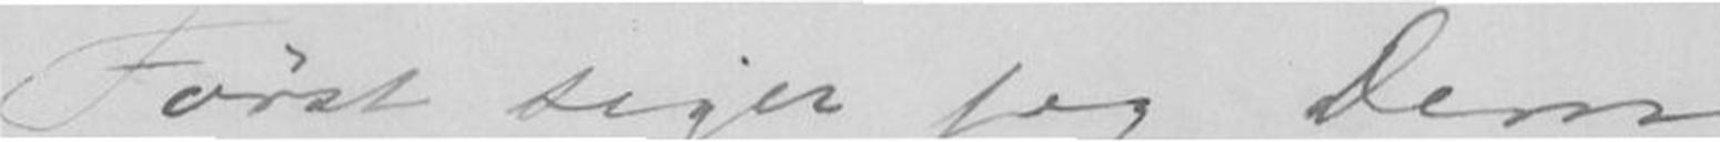Først siger jeg Dem
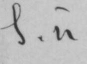S.u.
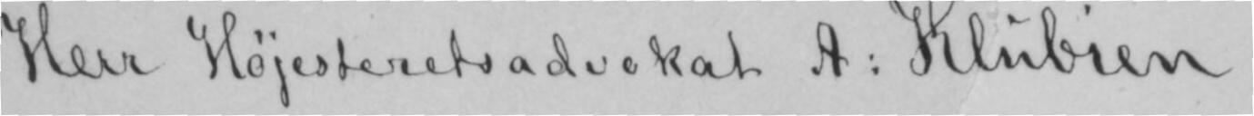Herr Højesteretsadvokat A: Klubien.
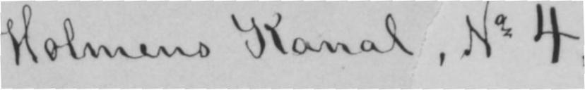Holmens Kanal, No 4.
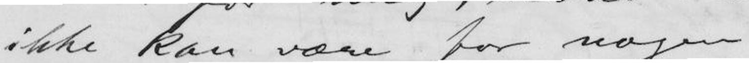ikke kan være for nogen
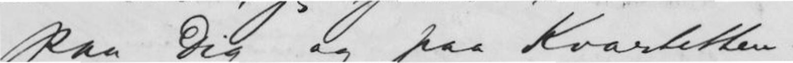paa Dig og paa Kvartetten!
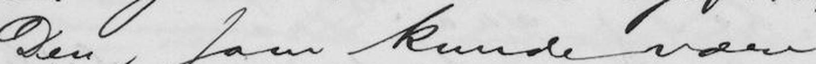- Den, som kunde være
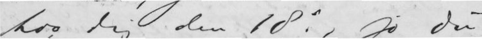hos Dig den 18de, jo du
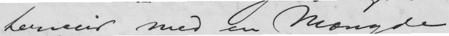terveir med en Mængde
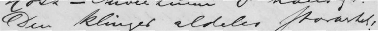Den klinger aldeles storartet!
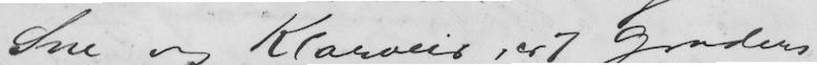Sne og Klarveir, ca 7 Graders
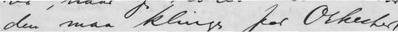den maa klinge for Orkester!
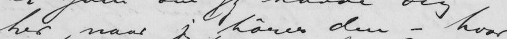her, naar jeg hører den - hvor
